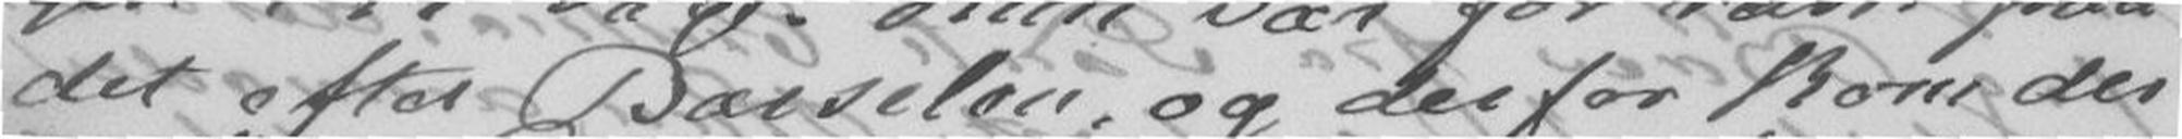det efter Barselen, og derfor kom der
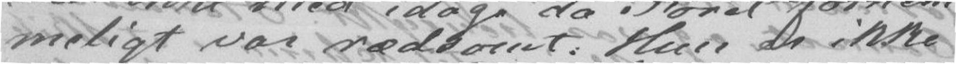meligt var rædsomt. Hun er ikke
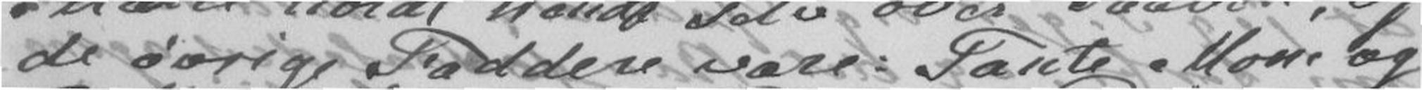de øvrige Faddere vare Tante Mone og
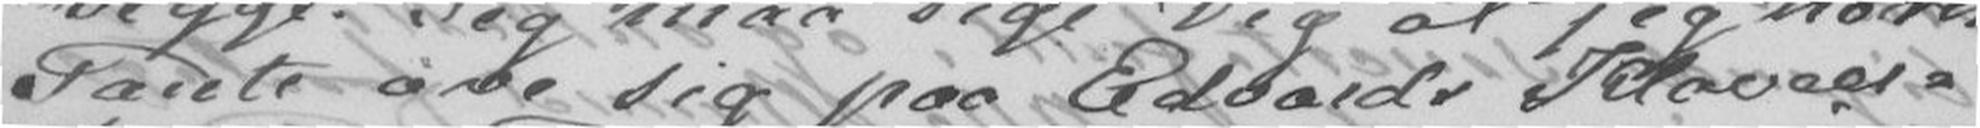Tante øve sig paa Edvards Klaveer-
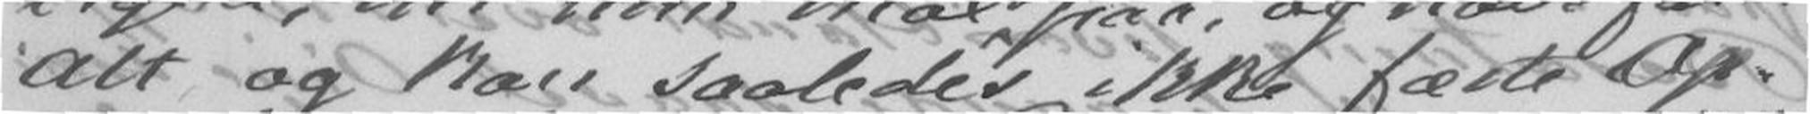Alt og kan saaledes ikke fæste Op-
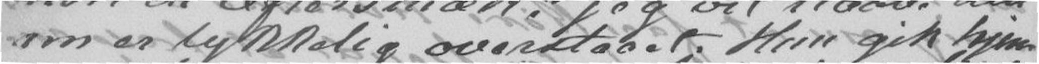nu er lykkelig overstaaet. Hun gik hjem
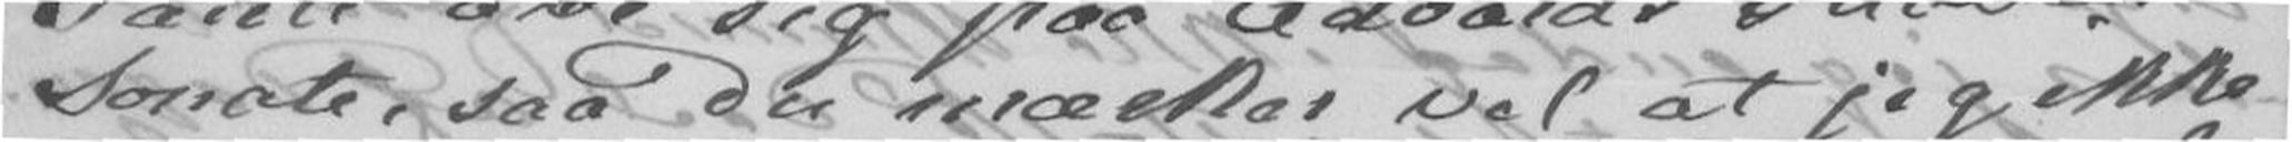Sonate, saa Du mærker vel at jeg ikke
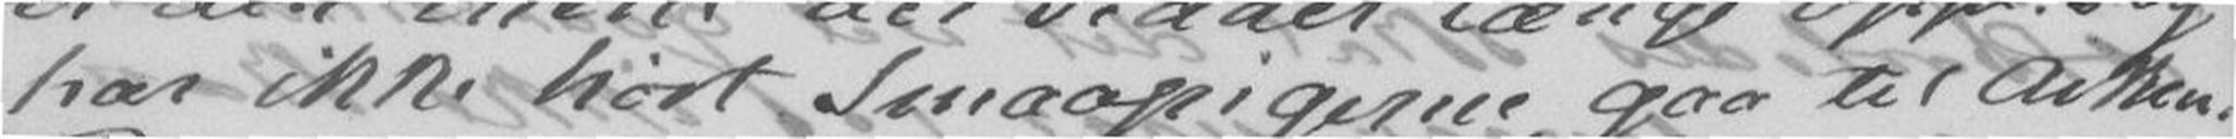har ikke hørt Smaapigerne gaa til Arken.
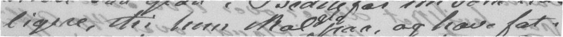ligere, thi hun skal paa, og have fat i
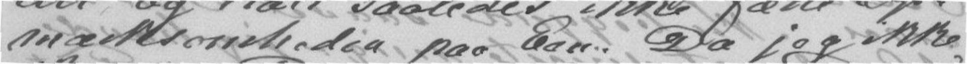mærksomheden paa Een. Da jeg ikke
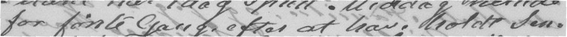for første Gang efter at have holdt Sen-
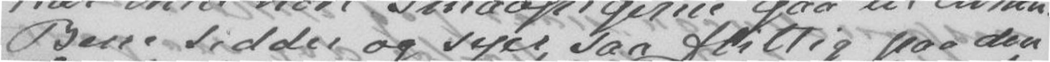Bene sidder og syer saa flittig paa den
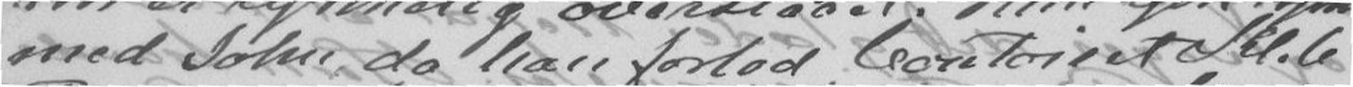med John, da han forlod Contoiret Kl.6
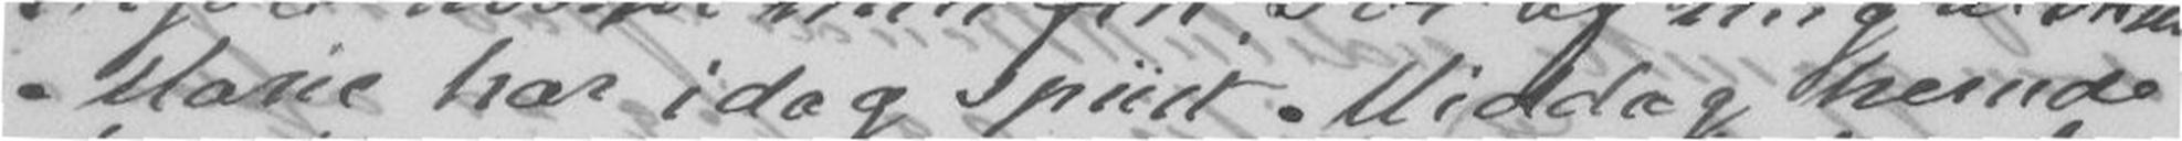Marie har idag spiist Middag herude
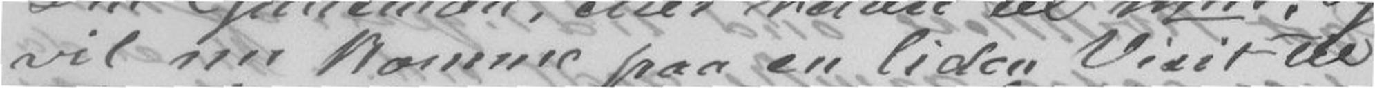vil nu komme paa en liden Visit til
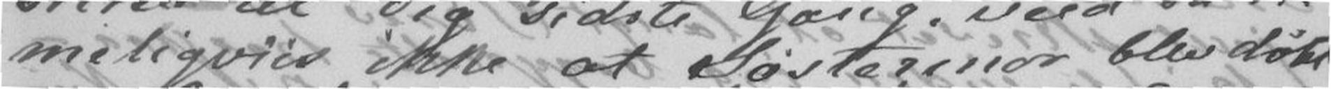meligviis ikke at Søstermor blev døbt
